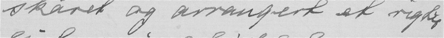skåret og arrangert et rigtig
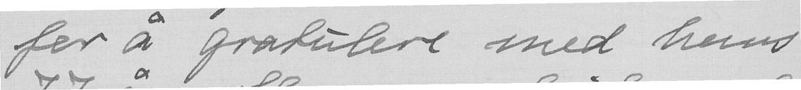for å gratulere med hans
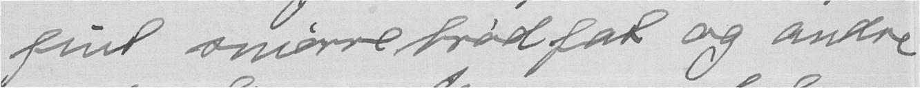fint smørrebrødfat og andre
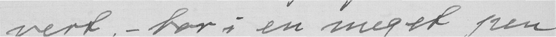vert, - bor i en meget pen
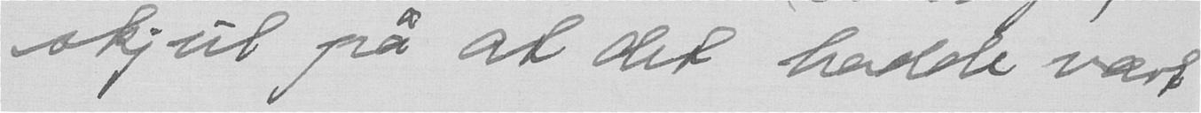skjul på at det hadde vært
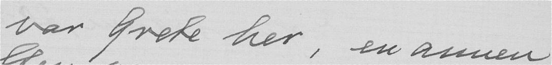var Grete her, en annen
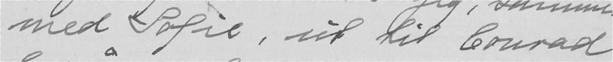med Sofie, ut til Conrad
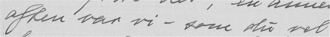aften var vi - som du vel
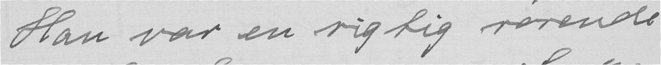Han var en rigtig rørende
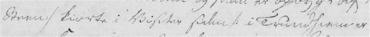Steen :/ kiørte i Visiter siden /: i Trundhiem er
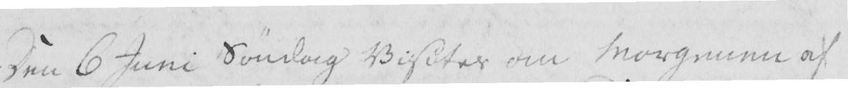Den 6 Juni Søndag Visiter om Morgenen af
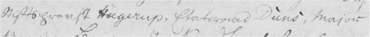Stiftsprovst Hagerup, Etatsraad Duns, Major
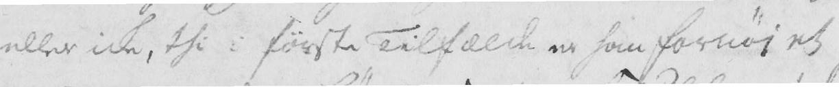eller icke, thi i første tilfælde er han fornøiet
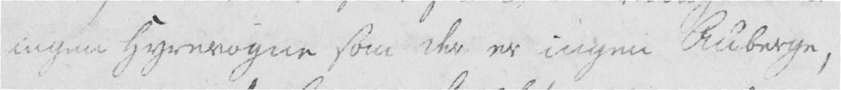ingen Hyrevogne som der er ingen Auberge,
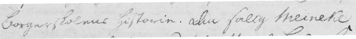Borgerskolens histore. Den salig Meincke
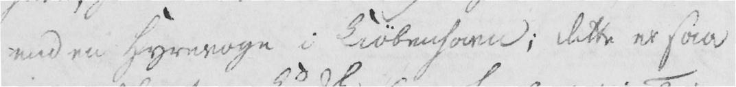end en Hyrevogn i Kiøbenhavn; dette er saa
